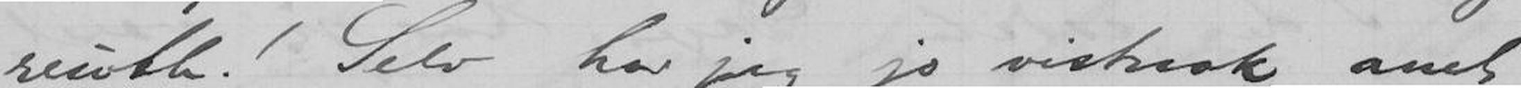reuth! Selv har jeg jo vistnok anet
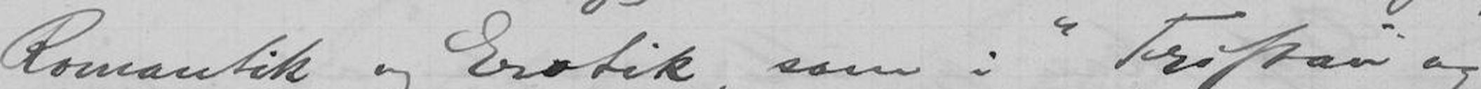Romantik og Erotik som i "Tristan" og
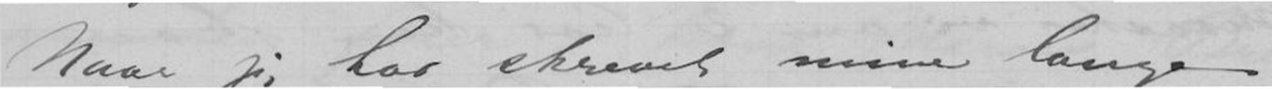Naar jeg har skrevet mine lange
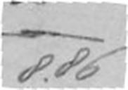8.86
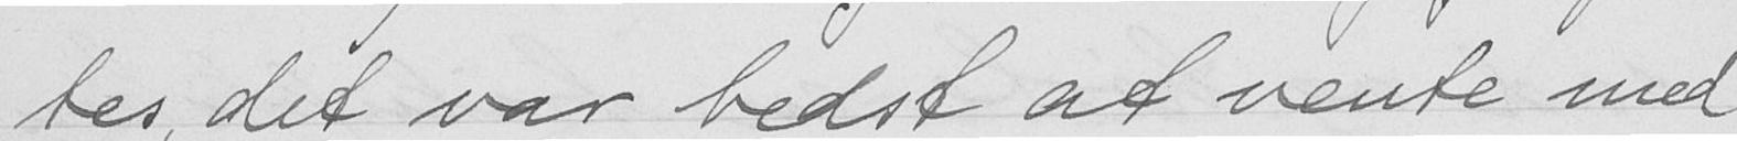tes, det var bedst at vente med
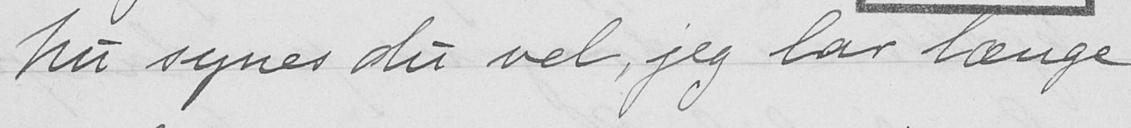Nu synes du vel, jeg lar længe
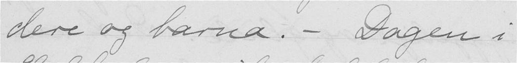dere og barna. - Dagen i
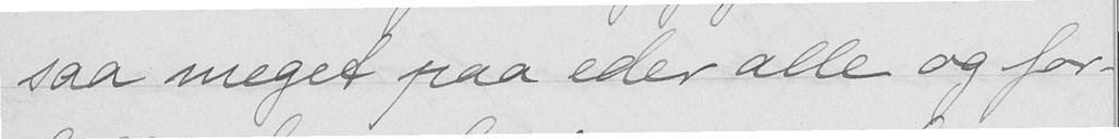saa meget paa eder alle og for-
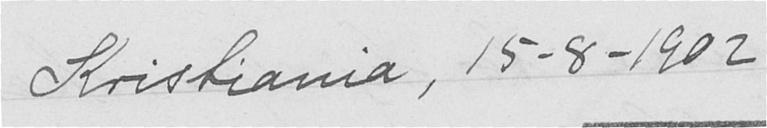Kristiania, 15-8-1902
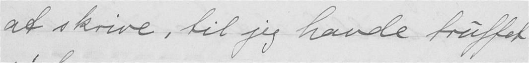at skrive, til jeg havde truffet
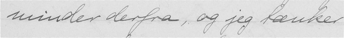minder derfra, og jeg tænker
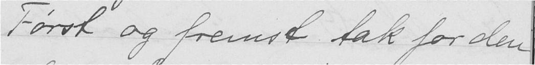Først og fremst tak for den
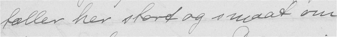tæller her stort og smaat om
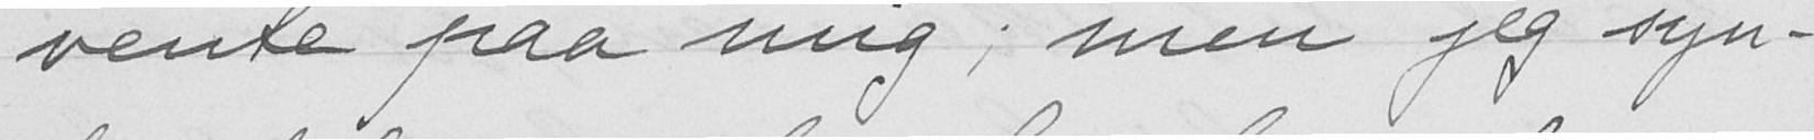vente paa mig; men jeg syn-
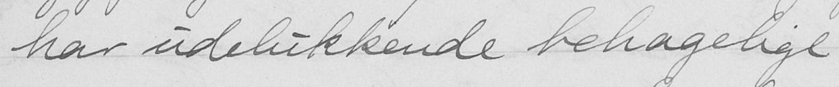har udelukkende behagelige
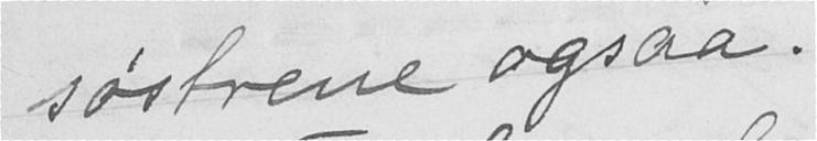søstrene ogsaa.
